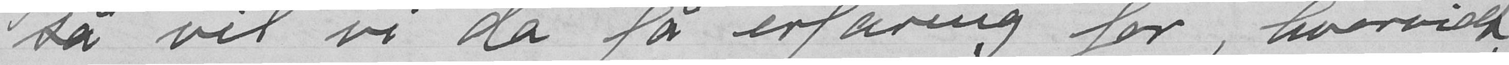så vil vi da få erfaring for, hvorvidt
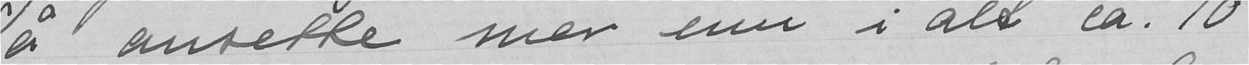å ansette mer enn i alt ca. 10
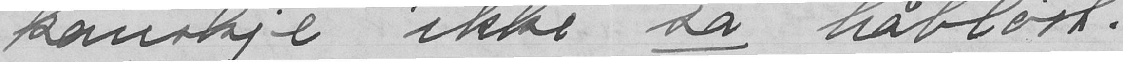kanskje ikke så håbløst.
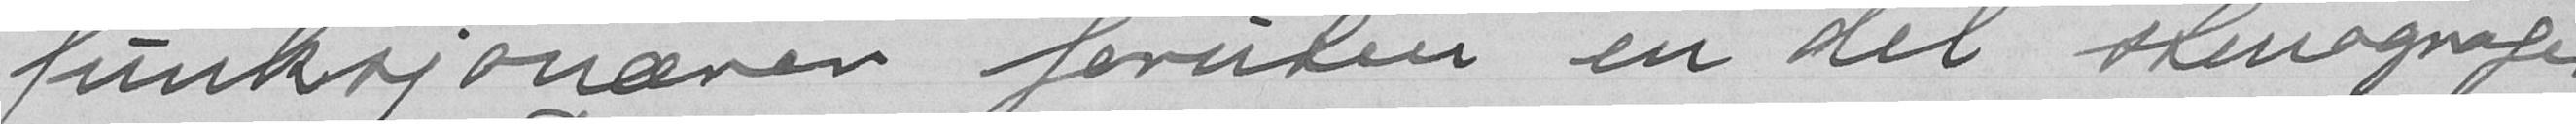funksjonærer foruten en del stenografer
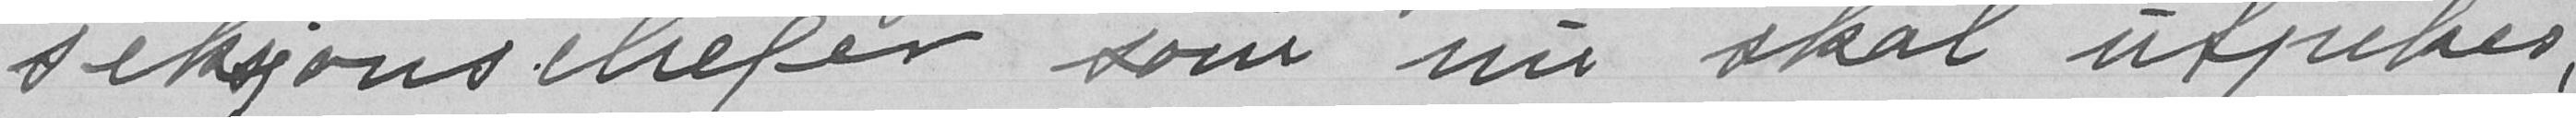seksjonschefer som nu skal utpekes,
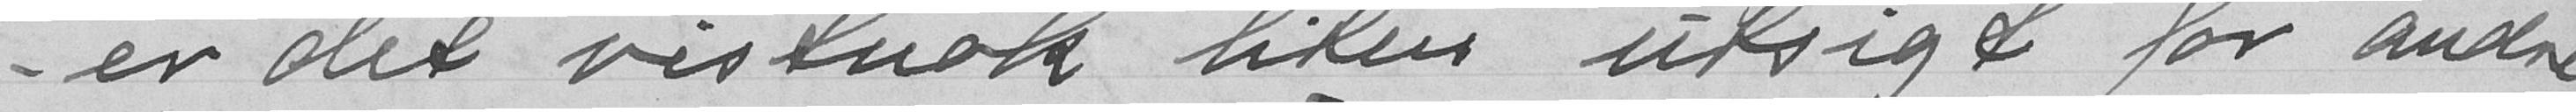- er det vistnok liten utsikt for andre
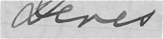Deres
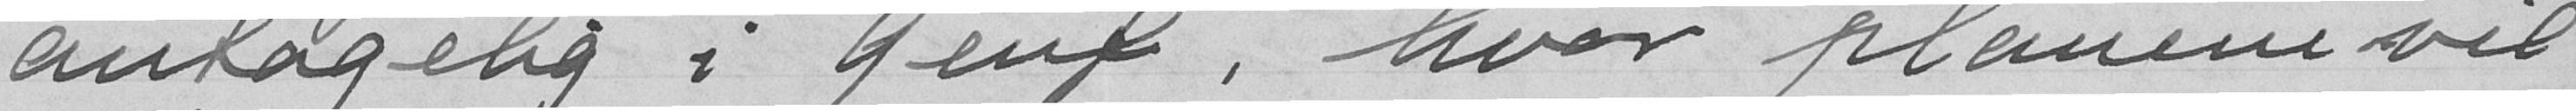antagelig i Gent, hvor planene vil
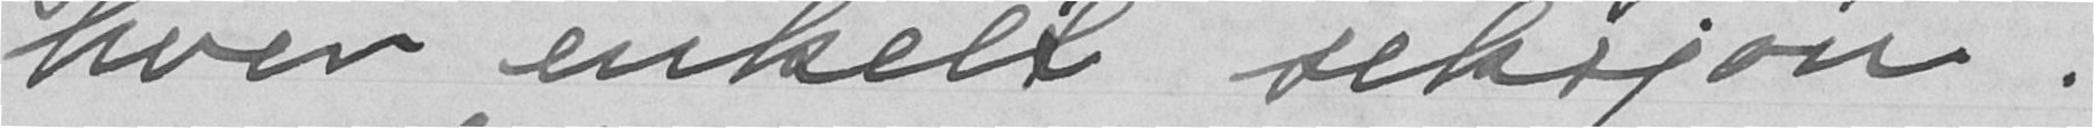hver enkelt seksjon.
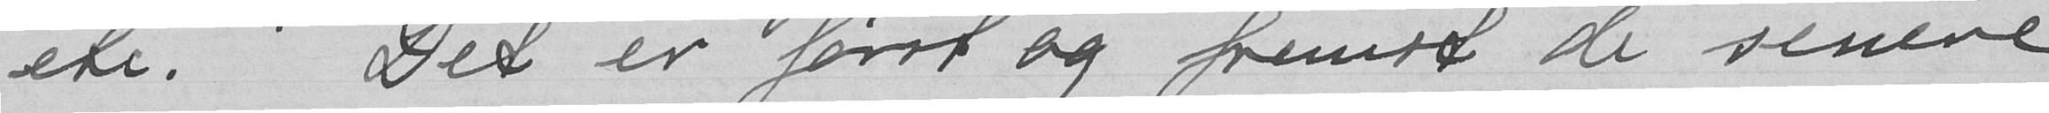etc. Det er først og fremst de senere
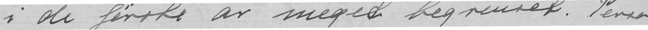i de første år meget begrenset. Perso-
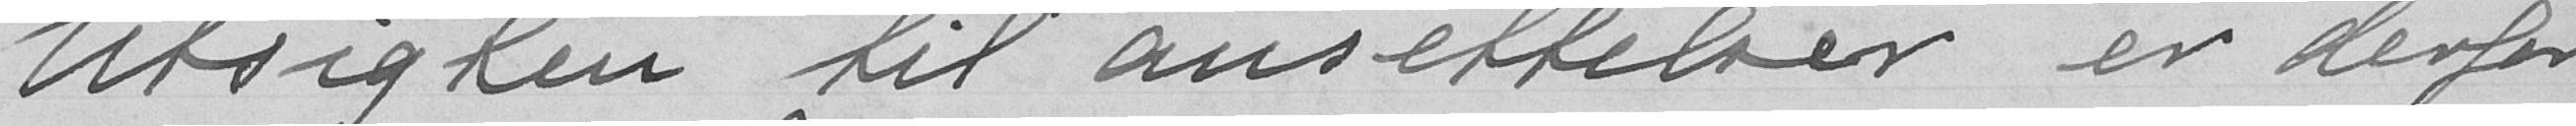Utsigten til ansettelser er derfor
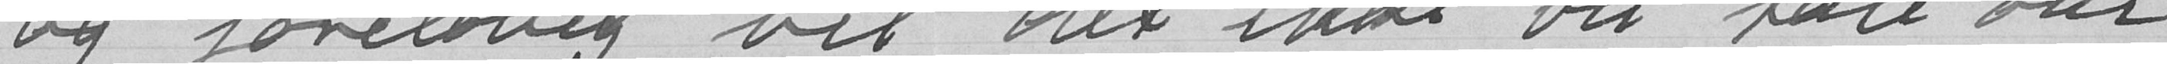og foreløbig vil det ikke bli tale om
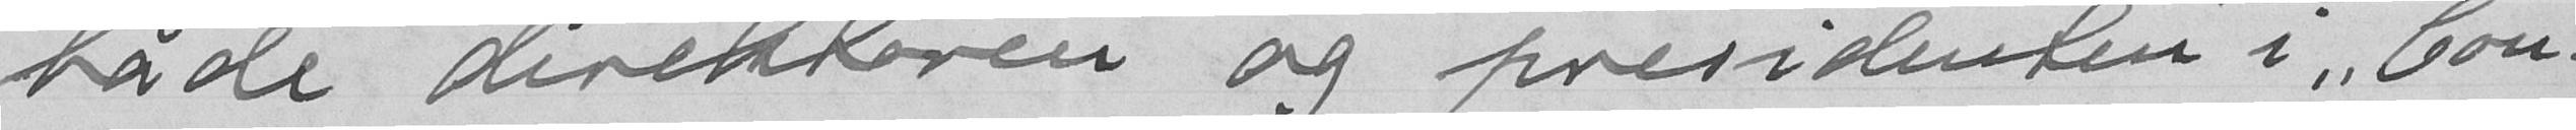både direktøren og presidenten i Con-
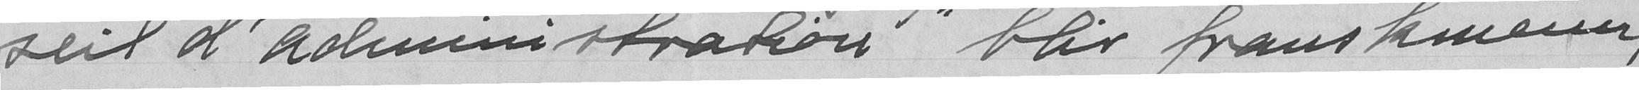seil d'administration blir franskmenn,
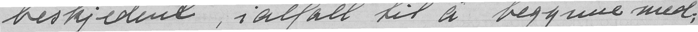beskjedent, ialfald til å begynne med,
